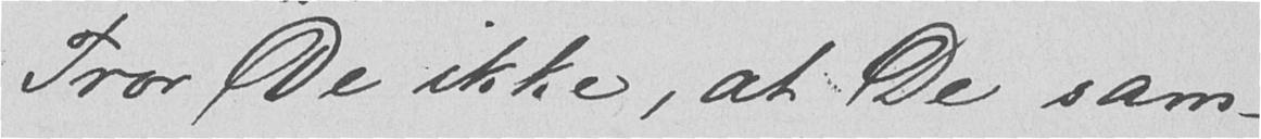Tror De ikke, at De sam-
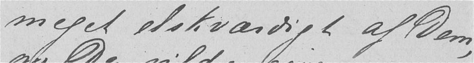meget elskværdigt af Dem,
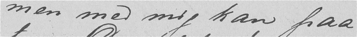men med mig kan paa-
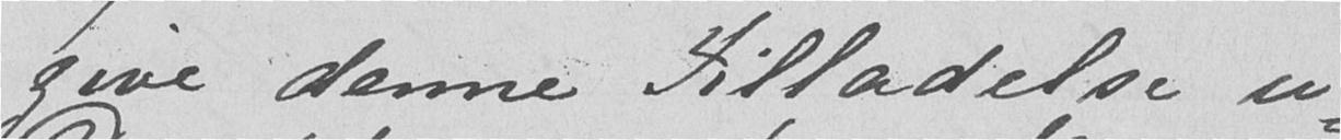give denne Tilladelse u-
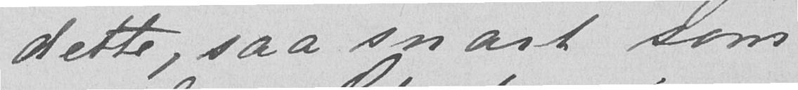dette, saa snart som
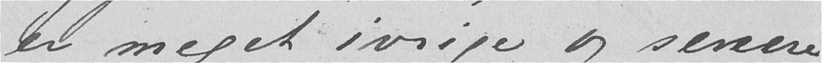er meget ivrige og senere
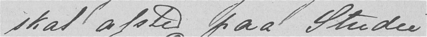skal afsted paa Studie-
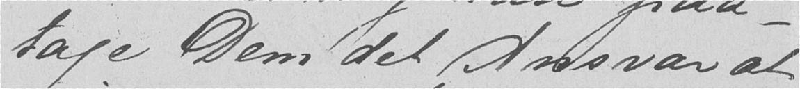tage Dem det Ansvar at
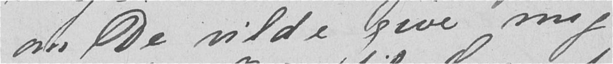om De vilde give mig
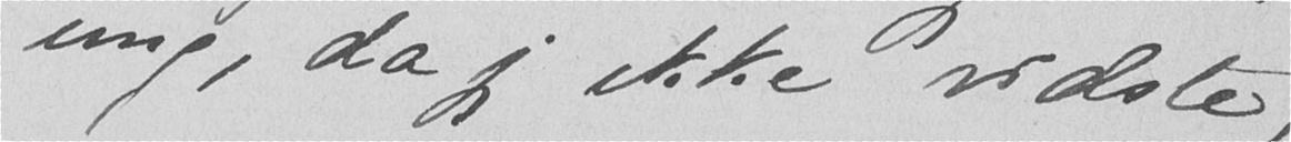ung, da jeg ikke vidste,
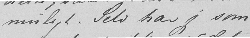muligt. Selv har jeg som
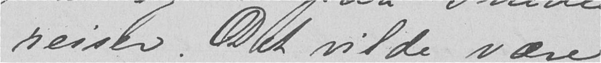reiser. Det vilde være
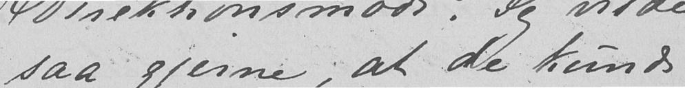saa gjerne, at de kunde
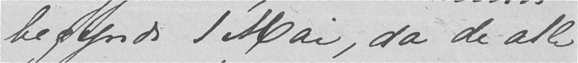begynde 1 Mai, da de alle
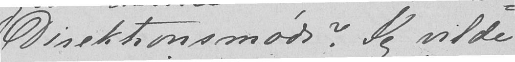den Direktionsmøde? Jeg vilde
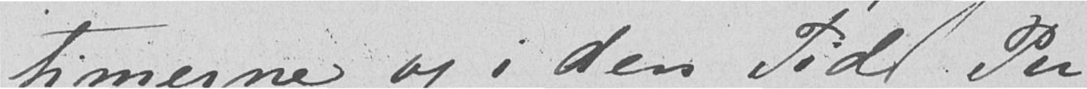timerne og i den Tid Pu-
